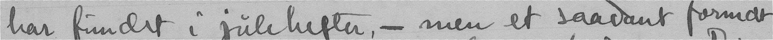har fundet i julehefter, - men et saadant format
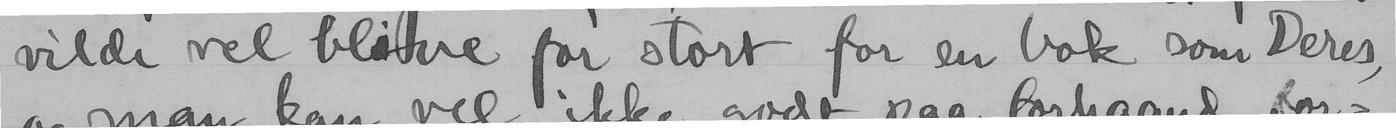vilde vel blive for stort for en bok som Deres,
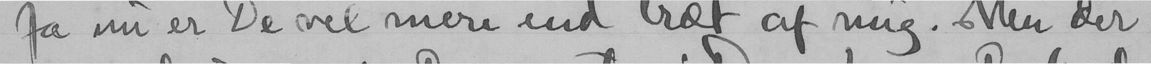Ja nu er De vel mere end træt af mig. Men der
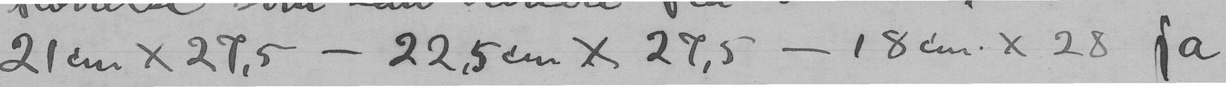21c. x 27,5 - 22,5cm x 27,5 - 18cm. x 28 fra
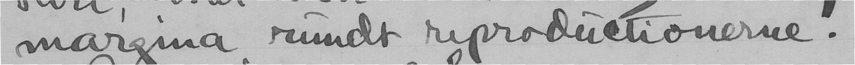margina rundt reproductionerne.
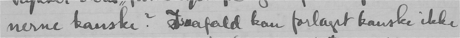nerne kanske? Isaafald kan forlaget kanske ikke
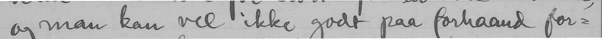og man kan vel ikke godt paa forhaand for-
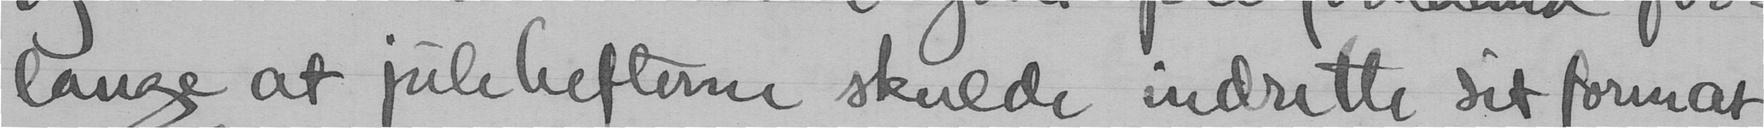lange at julehefterne skulde indrette sit format
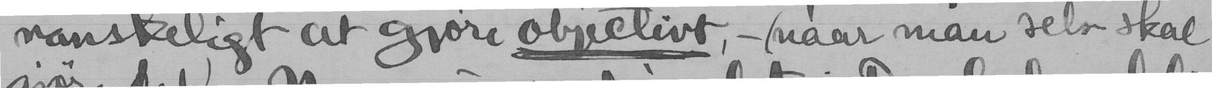vanskeligt at gjøre objectivt, - (naar man selv skal
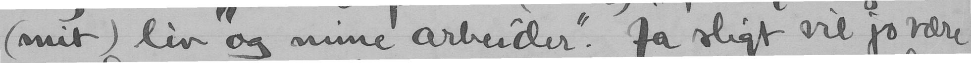(mit) liv og mine arbeider". Ja sligt vil jo være
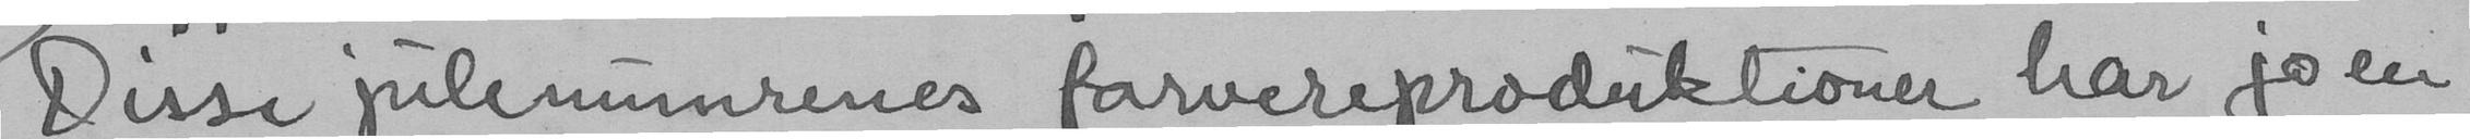Disse julenummrenes farvereproduktioner har jo en
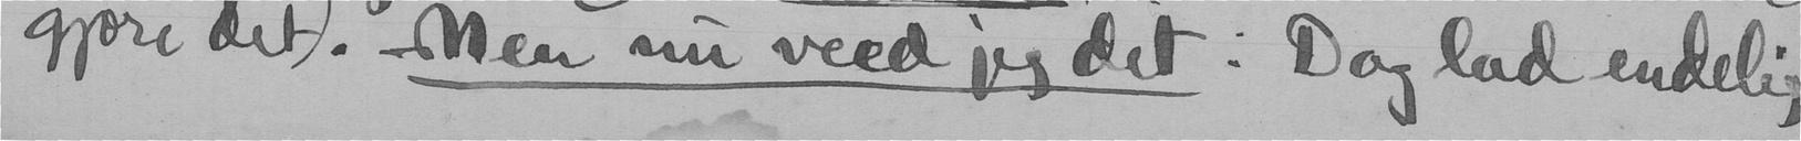gjøre det). Men nu veed jeg det: Dog lad endelig
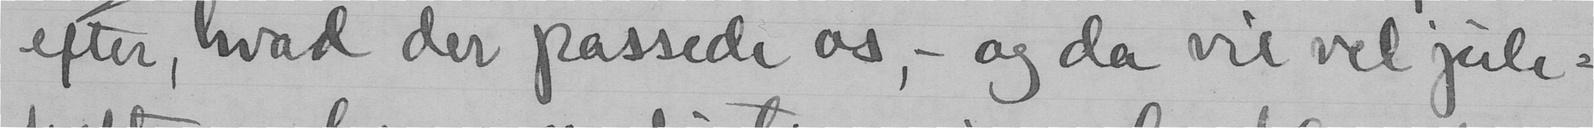efter, hvad der passede os - og da vil vel jule-
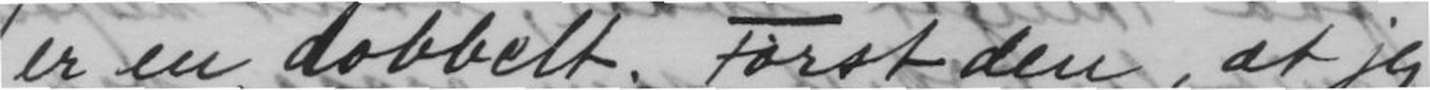er en dobbelt. Først den, at jeg
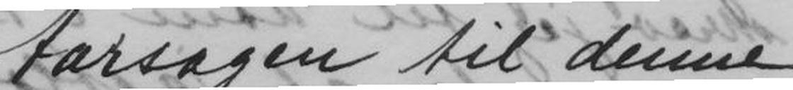Aarsaken til denne
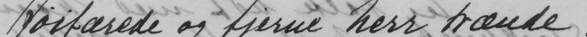Høitærede og fjerne herr Frænde!
